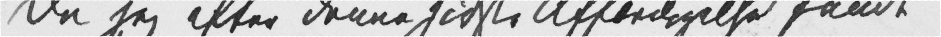Da jeg efter denne sidste Affærdigelse fandt
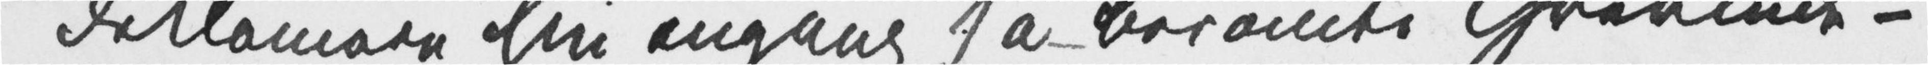deklamere hin engang så berømte Grevinde-
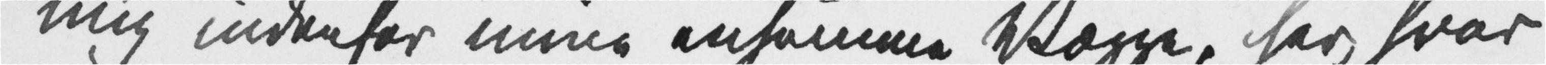mig indenfor mine ensomme Vægge, her, hvor
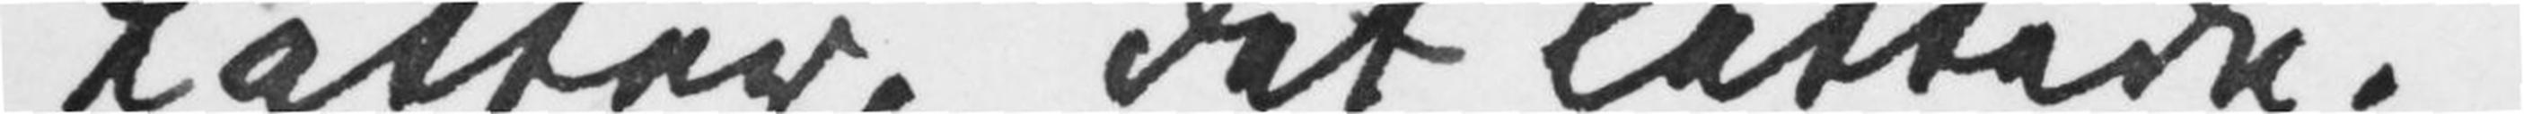Latter, det lettede.
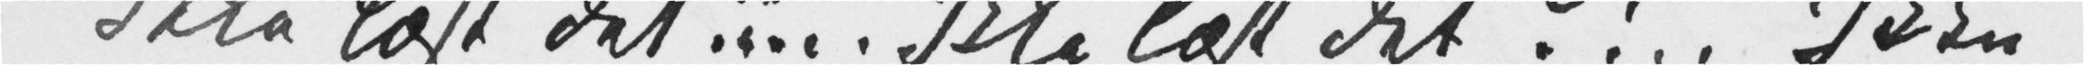«Ikke læst det!!... Ikke læst det?!.. Ikke
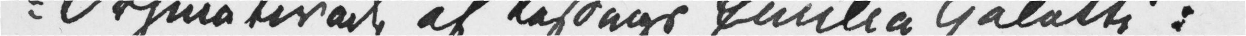Orsinatirade af Lessings Emilia Galotti:
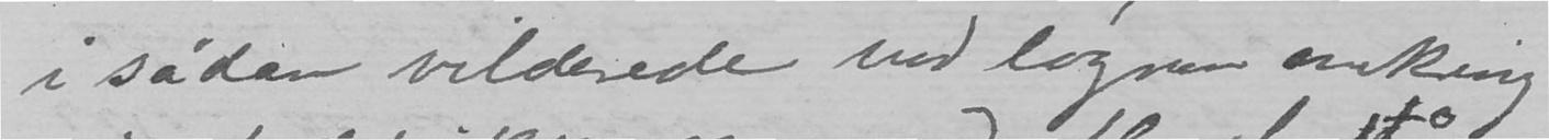i såden vilderede med løgnen omkring
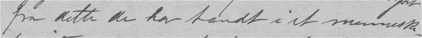fra dette de har tændt i et menneske-
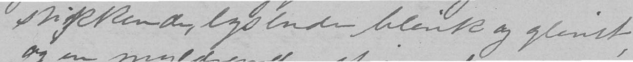stikkende, lysende blink og glimt,
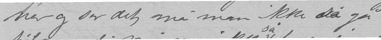ner og ser det, må man ikke så gå
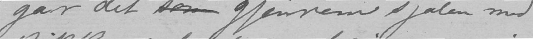går det  gjennem sjælen med
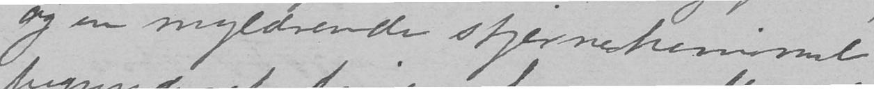og en myldrende stjernehimmel
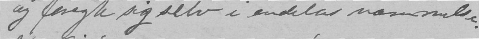og foragte sig selv i endeløs væmmelse.
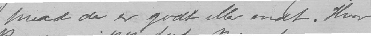hvad der er godt eller ondt. Hvor
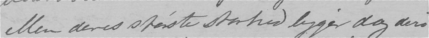eller deres største storhed ligger dog deri
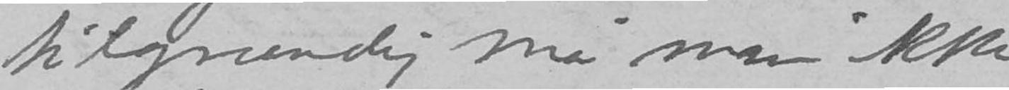tilgrunde; må man ikke
